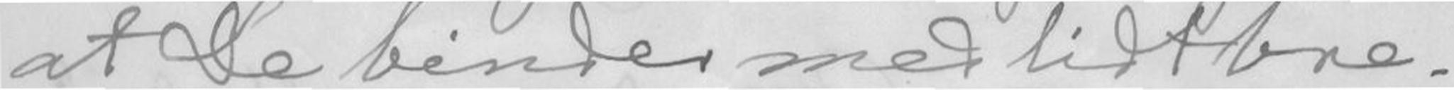at De binder med lidt bre-
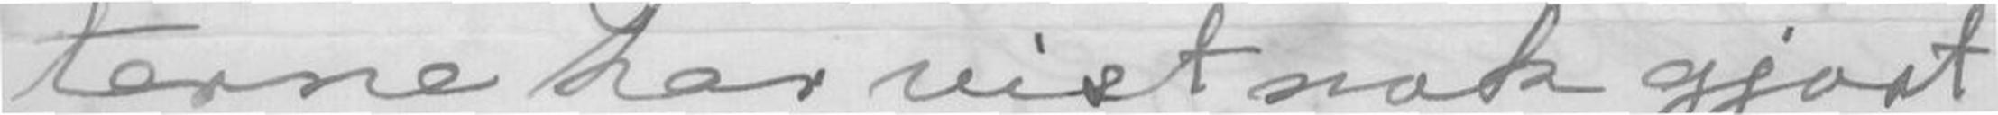terne har vist nok gjort
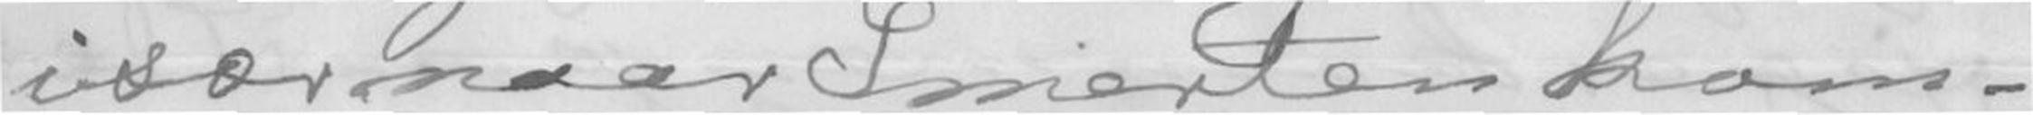især naar Smerten kom-
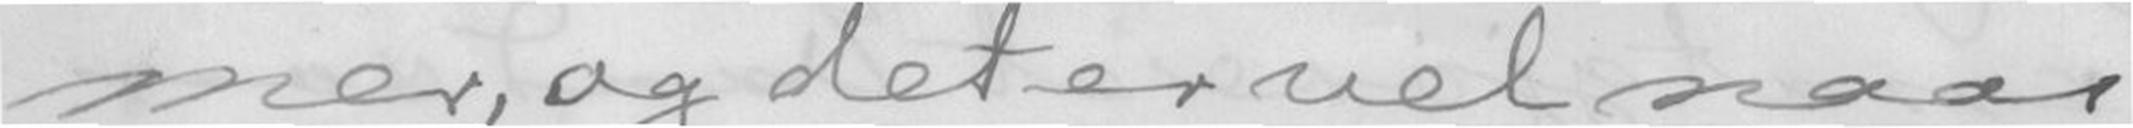mer, og det er vel naar
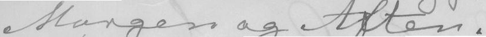Morgen og Aften.
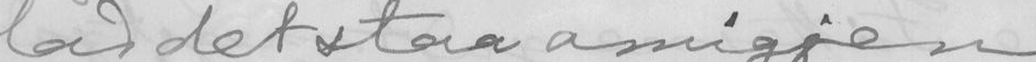lad det staa omigjen
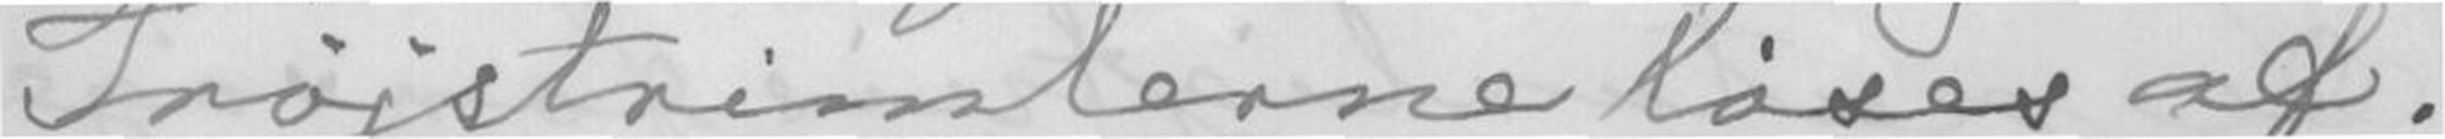Trøjstrimlerne løses af,
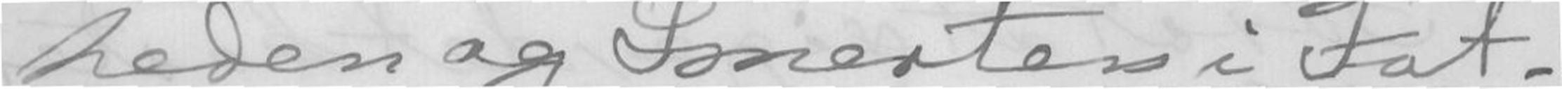heden og Smerten i Føt-
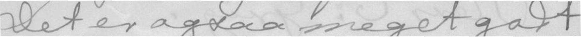Det er ogsaa meget godt
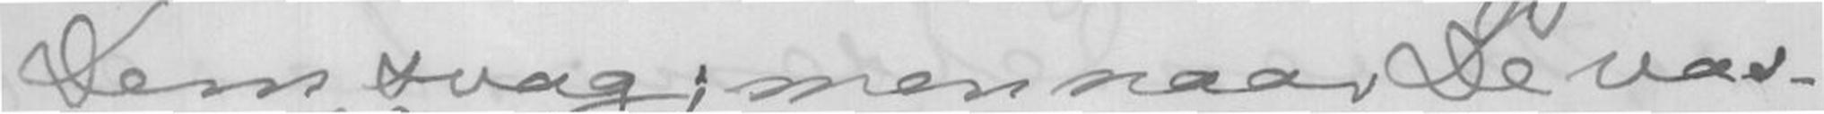Dem svag; men naar de vas-
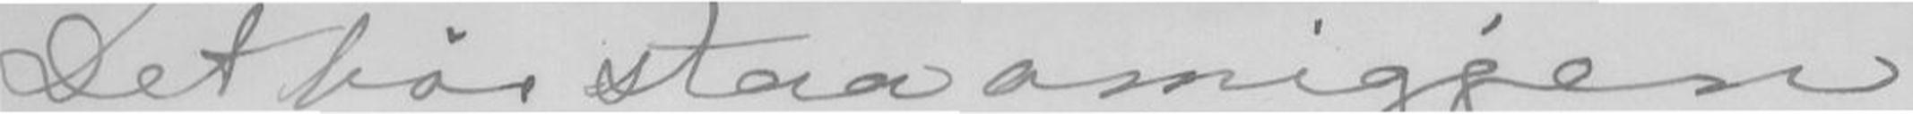Det bør staa omigjen
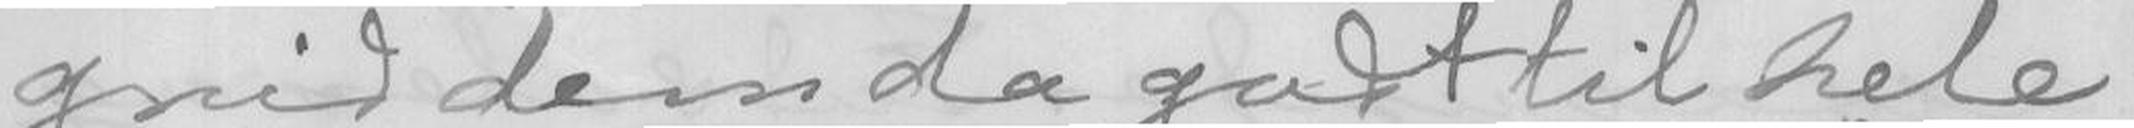gnid dem da godt til hele
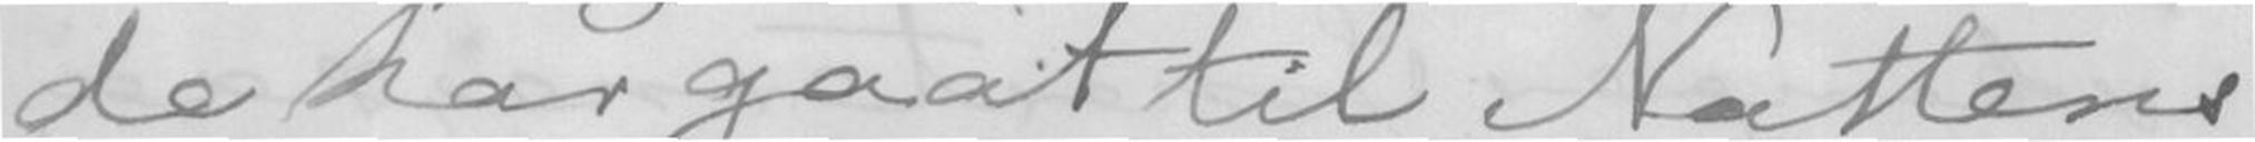de har gaat til Nattens
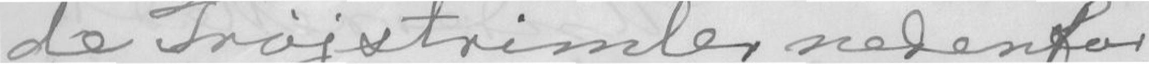de Trøjstrimler nedenfor
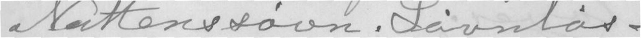Natterssøvn. Søvnløs-
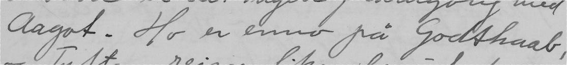Aagot. Ho er enno på Godthaab,
